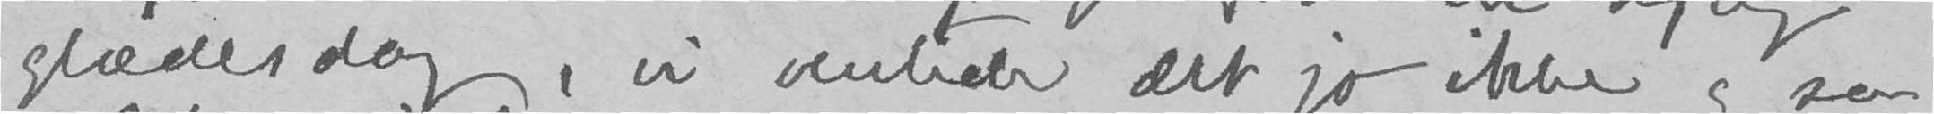glædesdag, vi ventede det jo ikke, og saa
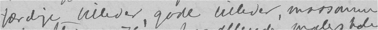færdige billider, gode billeder, morsomme
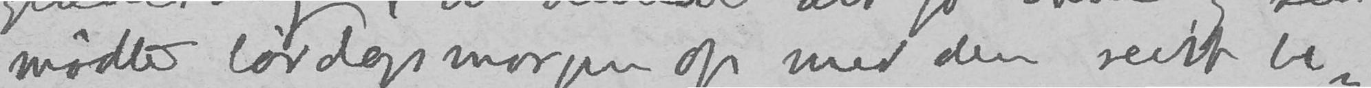mødte lørdagsmorgen op med den reitt be-
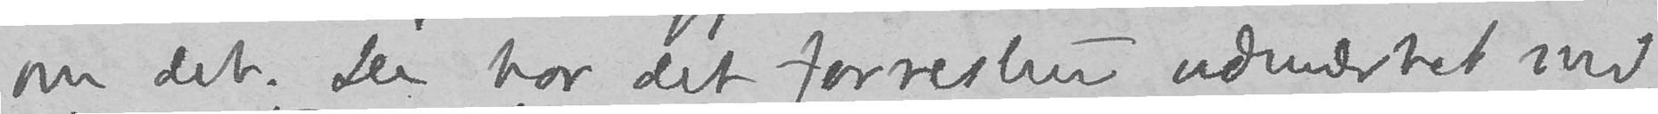om det. De har det forresten udmærket med
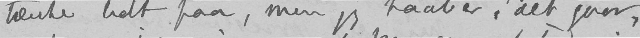tænke lidt paa, men jeg haaber, det gaar,
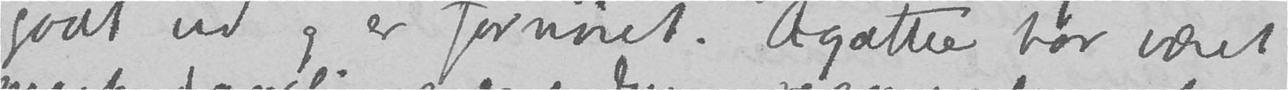godt ud og er fornøiet. Agathe har været
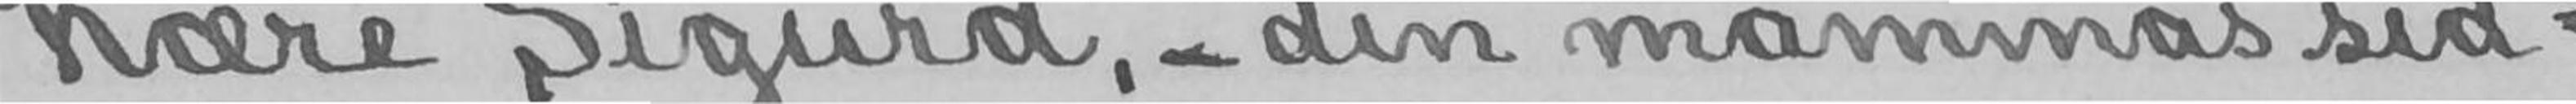Kære Sigurd,- din mammas sid-
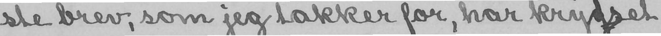ste brev, som jeg takker for, har krygdset
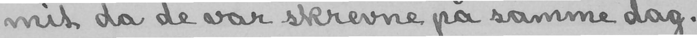mit da de var skrevne på samme dag.
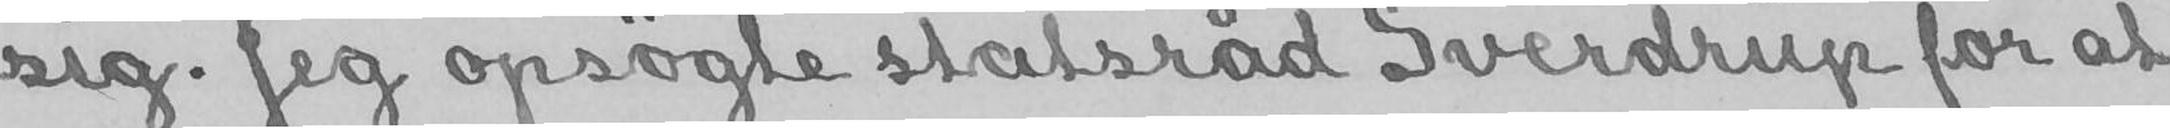sig. Jeg opsøgte statsråd Sverdrup for at
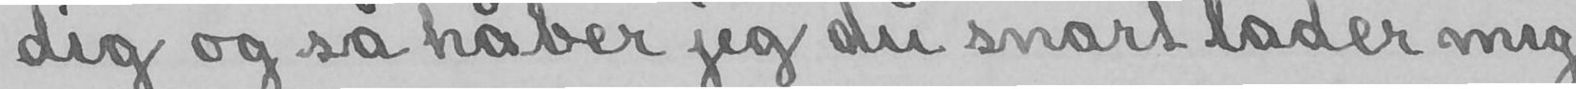dig og så håber jeg du snart lader mig
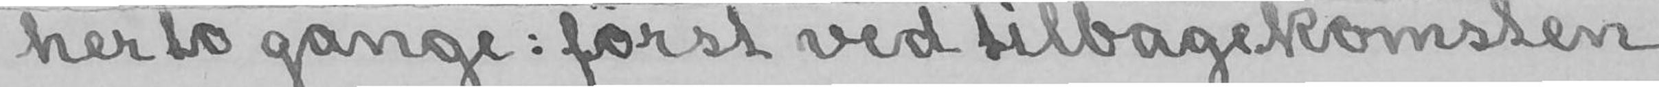her to gange: først ved tilbagekomsten
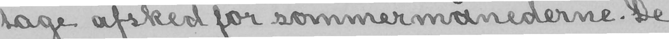tage afsked for sommermånederne. De
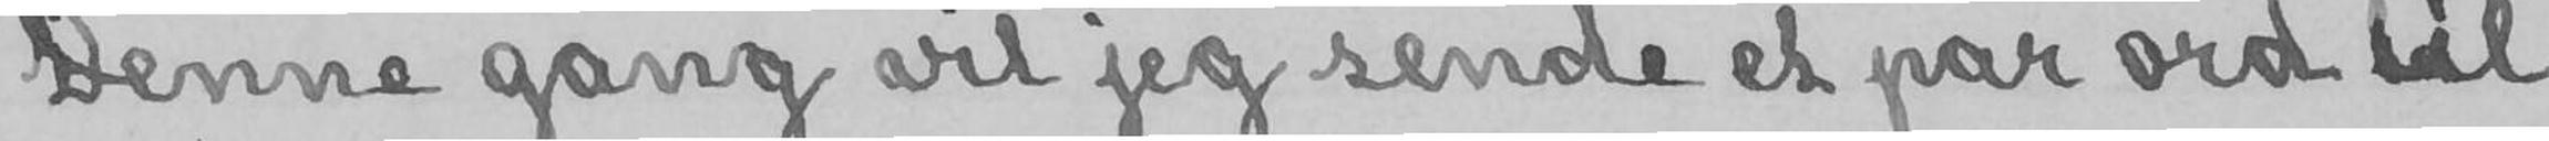Denne gang vil jeg sende et par ord til
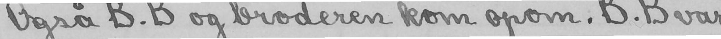Også B. B og broderen kom opom. B. B var
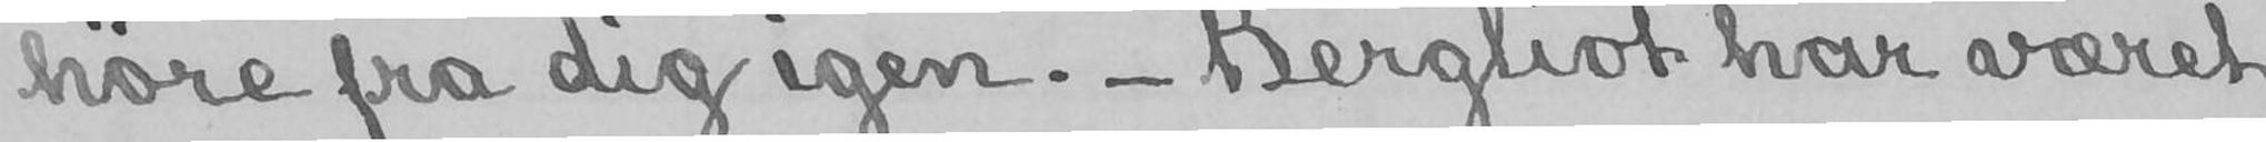høre fra dig igen.- Bergliot har været
